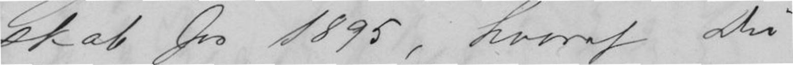skab for 1895, hvoraf Du
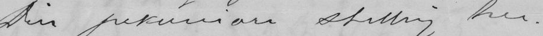Din pekuniære Stilling her.
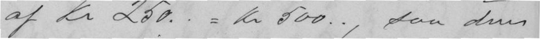af kr 250.- = kr 500.-, saa deres
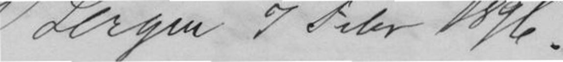Bergen 7 Febr 1896.
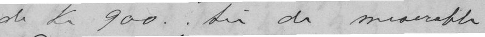de kr 900. til de miserable
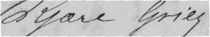Kjære Grieg!
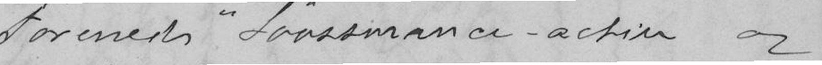Forende Søassurence-actier og
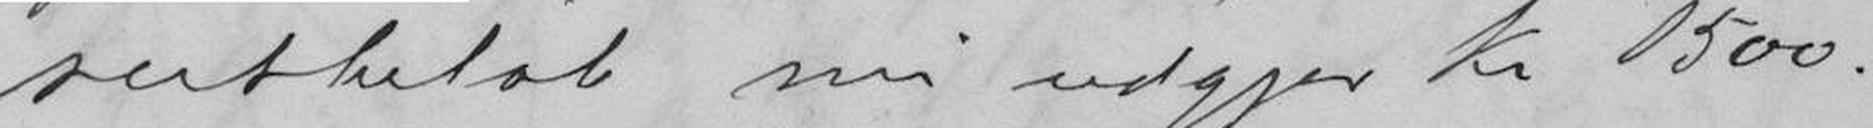restbeløb nu udgjør Kr 1500.
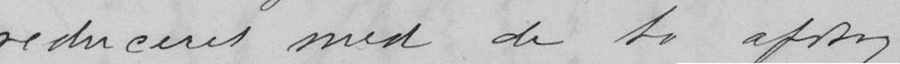reduceret med de to afdrag
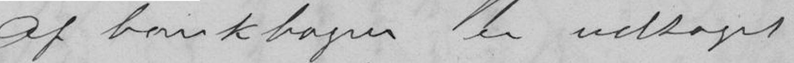Af bankbogen er udtaget
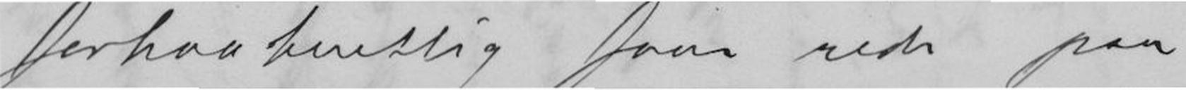forhaabentlig faar rede paa
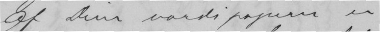Af Dine verdipapirer er
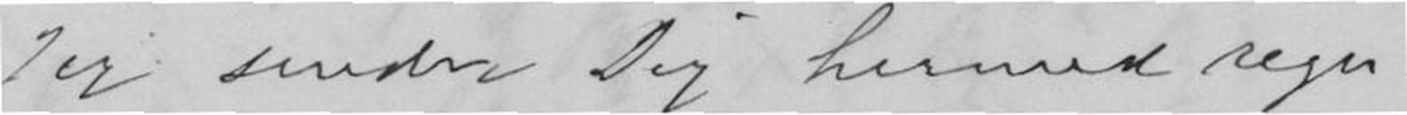Jeg sender Dig hermed regn-
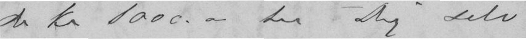de kr 1000.- til Dig selv.
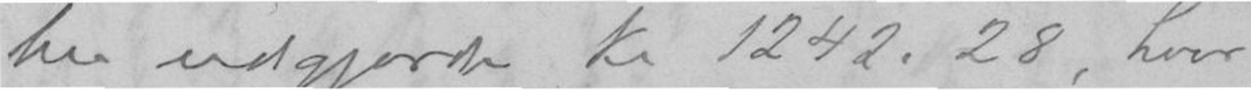ber udgjorde kr 1242.28, hvor
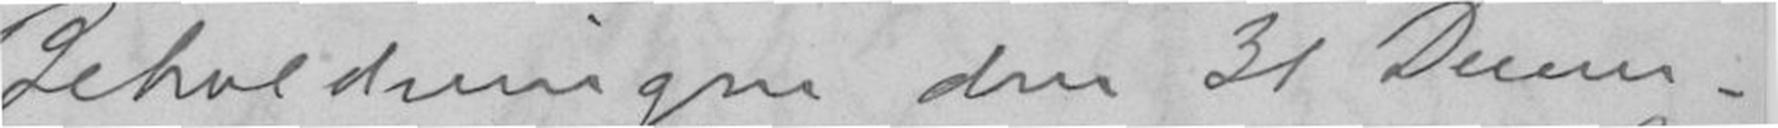Beholdningen den 31 Decem-
